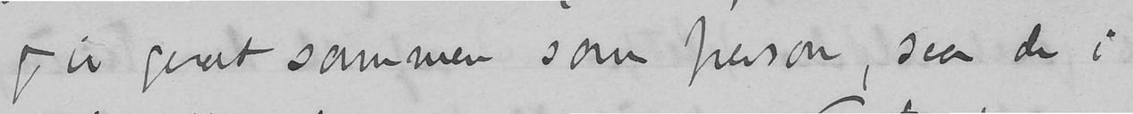vi groet sammen som person, saa de i
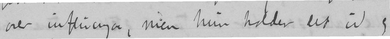over influenzae, men hun holder det ud og
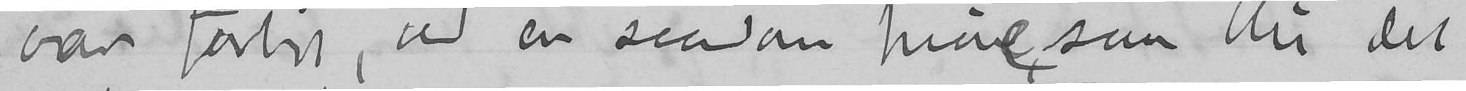var forbi, ved en saadan prie, saa blir det
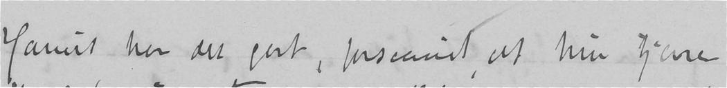Harriet har det godt, forsaavidt at hun tjener
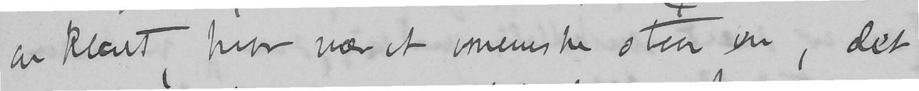en klart, hvor nær et menneske staar en, det
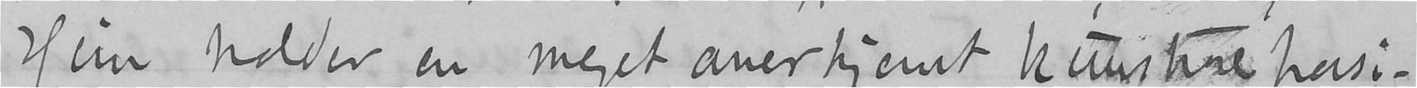Hun holder en meget anerkjendt kunstnerposi-
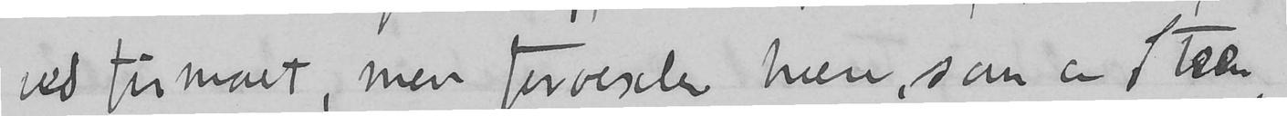ved firmaet, men forvexle hvem, som er Steen,
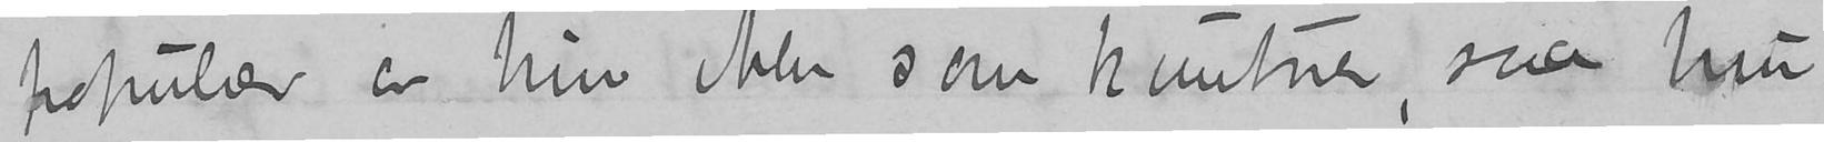populær er hun ikke som kuntner, saa hun
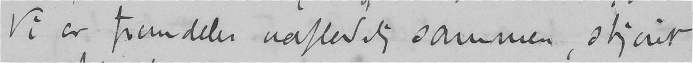Vi er fremdeles uafladelig sammen, skjønt
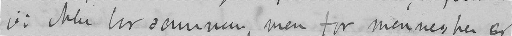vi ikke bor sammen, men for mennesker er
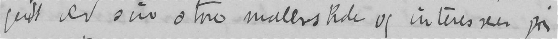godt ved sin store malerskole og interesserer sig
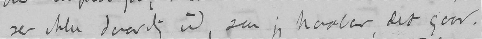ser ikke daarlig ud, saa jeg haaber, det gaar.
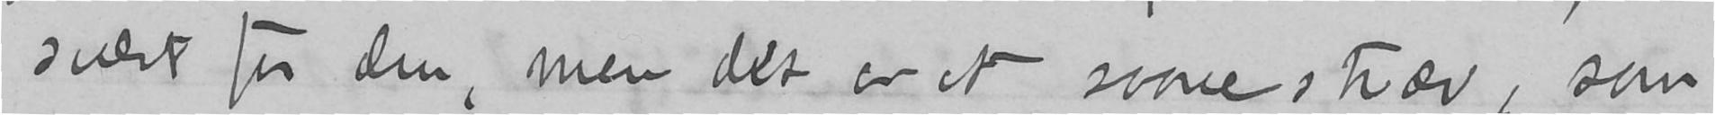svært for den, men det er et svare stræv, som
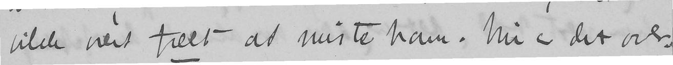vilde vært fælt at miste ham. Nu er det over.
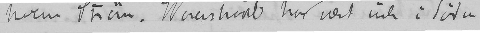hvem Strøm. Werenskiold har vært ude i lørda-
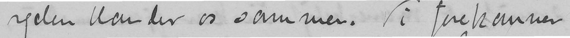regelen blander os sammen. Vi forekommer
